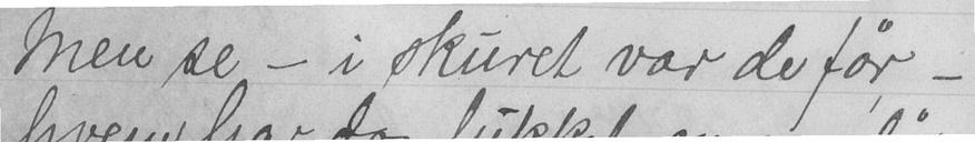Men se - i skuret var de før, -
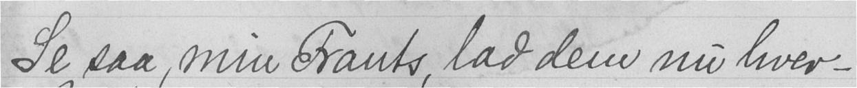Se saa, min Frants, lad dem nu hver -
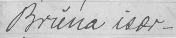Bruna især -
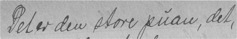Det er den store puan, det,
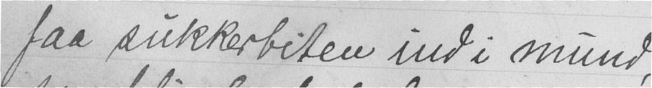faa sukkerbiten ind i mund,
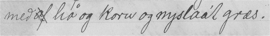med af hø og korn og nyslaa't græs.
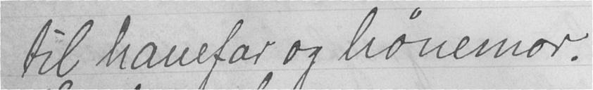til hanefar og hønemor.
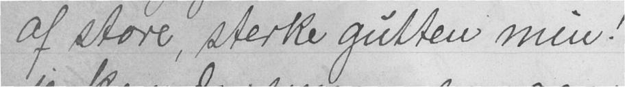af store, sterke gutten min!
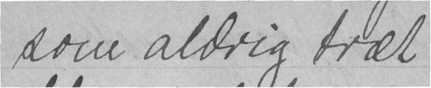som aldrig træt
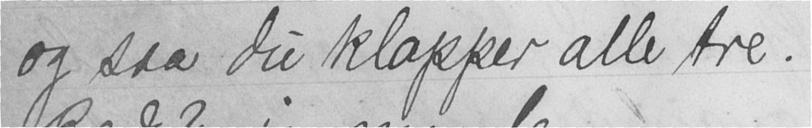og saa du klapper alle tre.
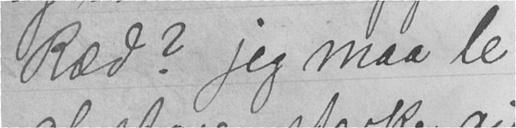Ræd? jeg maa le
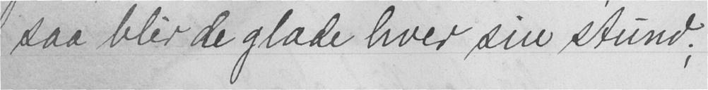saa blir de glade hver sin stund;
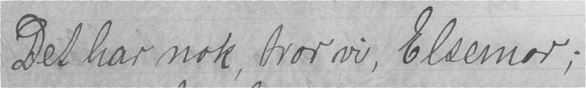Det har nok, tror vi, Elsemor;
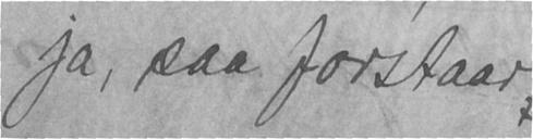ja, saa forstaar,
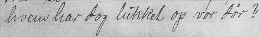hvem har dog lukket op vor dør?
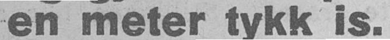en meter tykk is.
